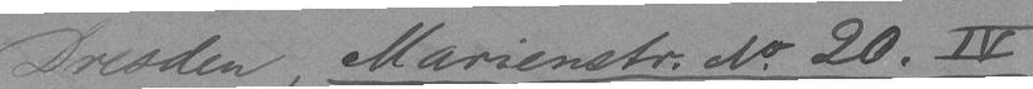Dresden, Marienstr. N. 20 IV
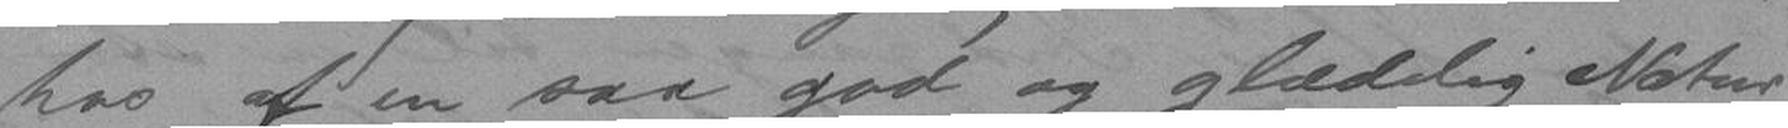has af en saa god og glædelig Natur,
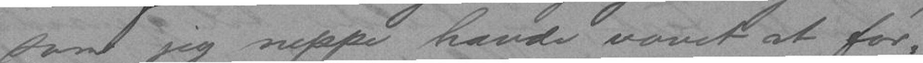som jeg neppe havde vovet at for-
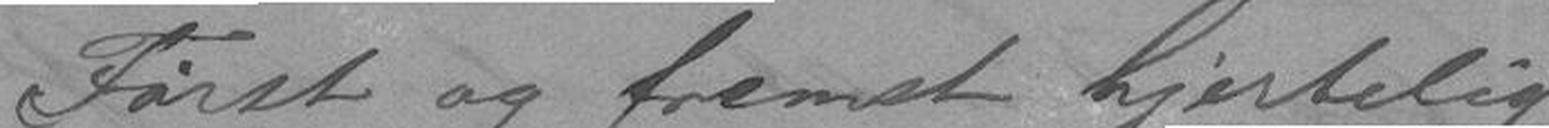Først og fremst hjertelig
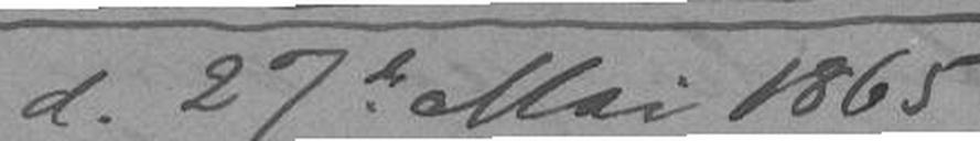d. 27de Mai 1865
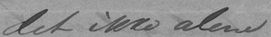det ikke alene
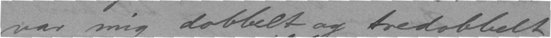var mig dobbelt og tredobbelt
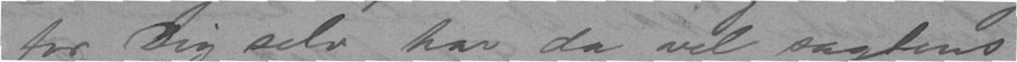for Dig selv har da vel sagtens
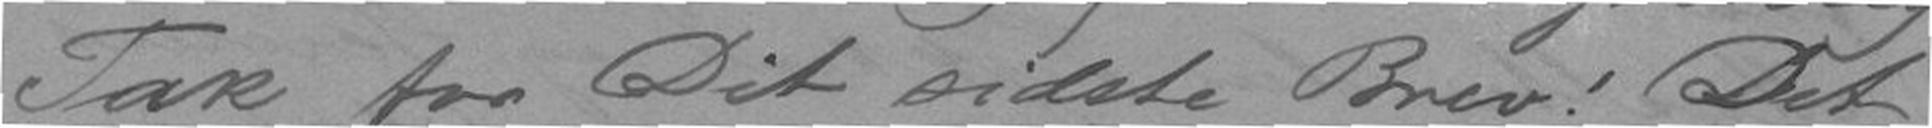Tak for Dit sidste Brev! Det
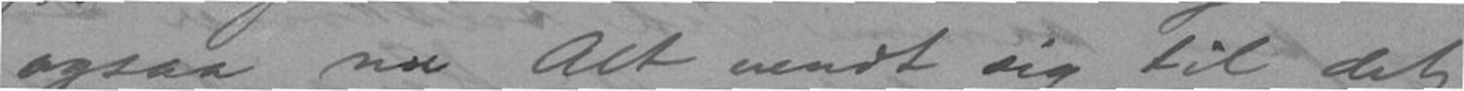ogsaa nu Alt vendt sig til det
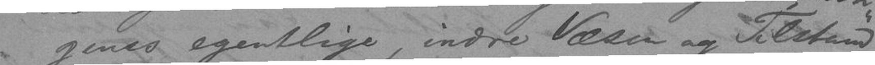genes egentlige, indre Væsen og Tilstand
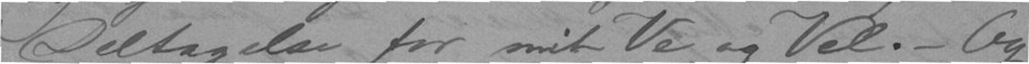Deltagelse for mit Ve og Vel. - Og
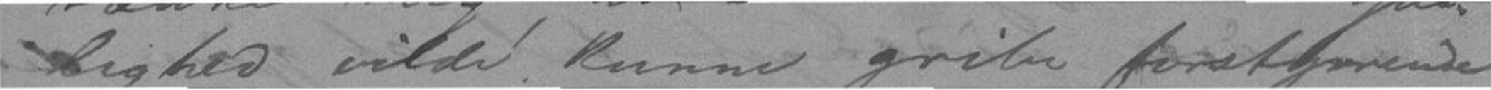lighed vilde kunne gribe forstyrrende
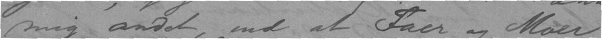mig andet, end at Faer og Moer
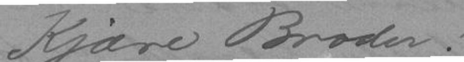Kjære Broder!
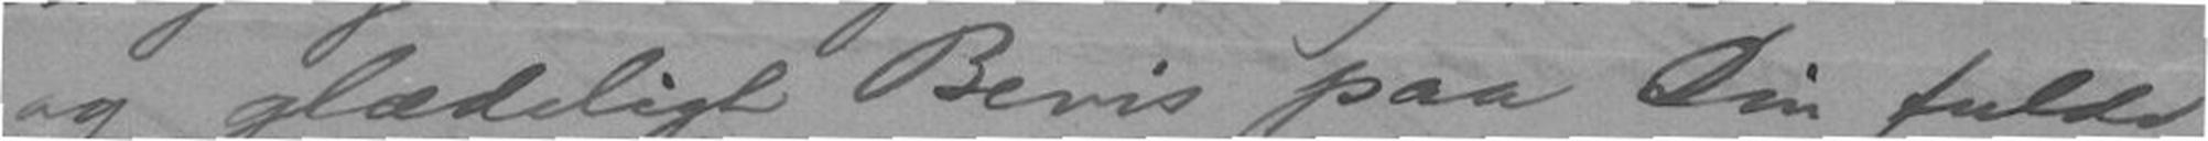og glædeligt Bevis paa Din fulde
